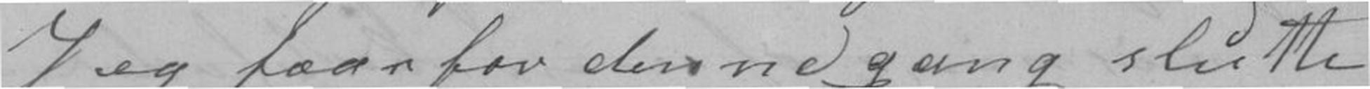Jeg faar for denne gang slutte
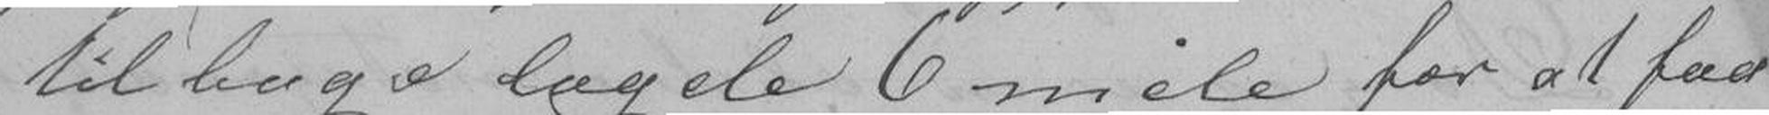til bage lagde 6 mile for at faa
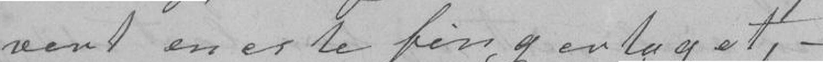vent eneste fingertaget,
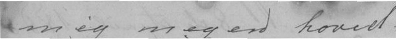mig megen hoved
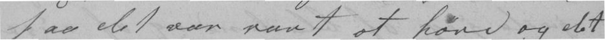saa det var rart at høre og det
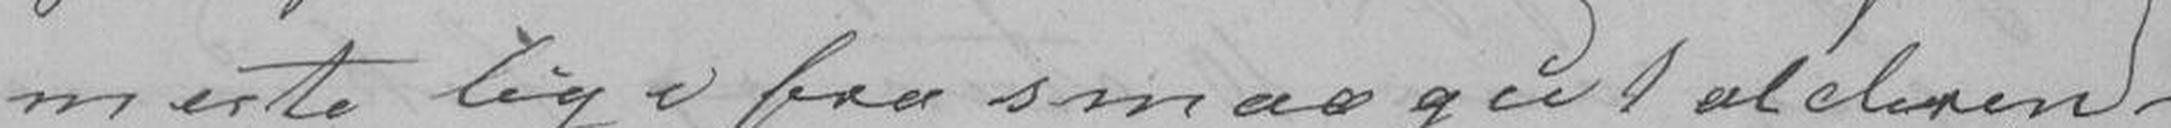meste lige fra smaagutalderen -
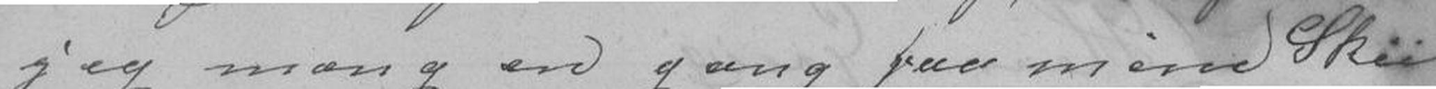jeg mang en gang paa mine Skü
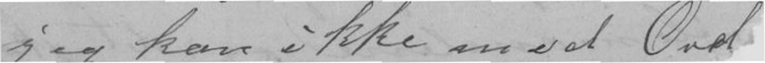jeg kan ikke med Ord.
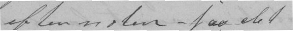efter noter - saa det
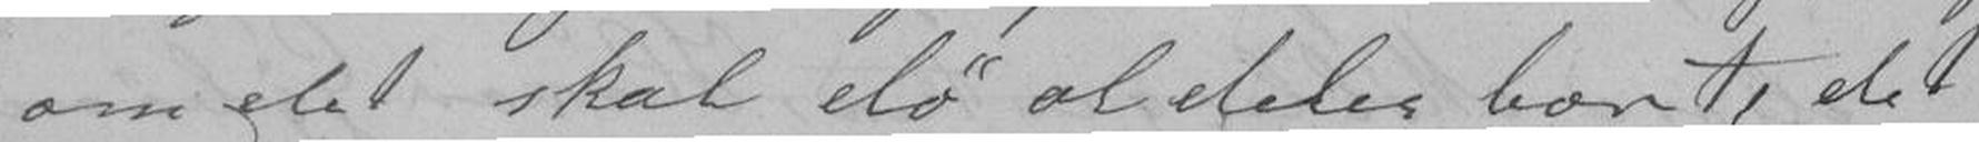om det skal dø aldeles bort, det
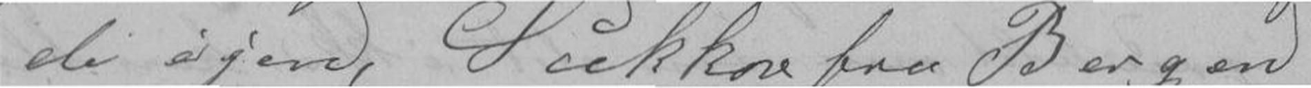de ijen, Sukkov fra Bergen
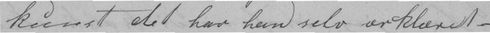kunst det har han selv ærklæret - -
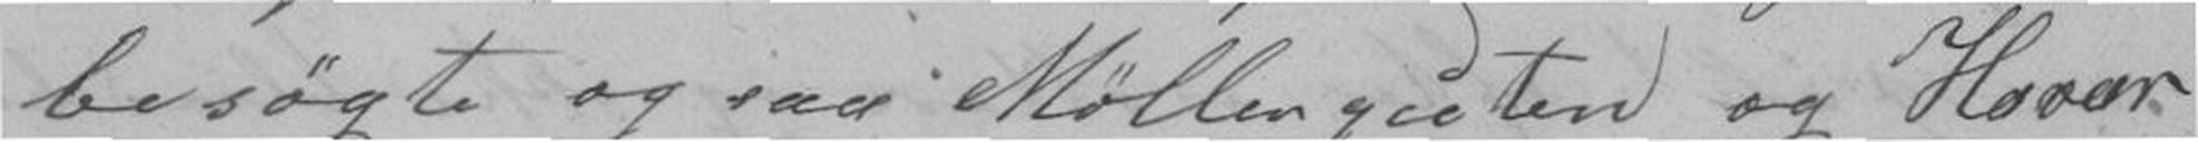besøgte ogsaa Møllerguten og Havar
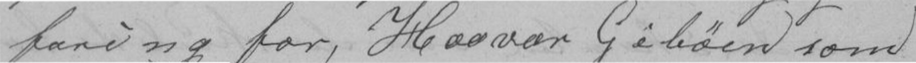faring for, Haavar Gibøen som
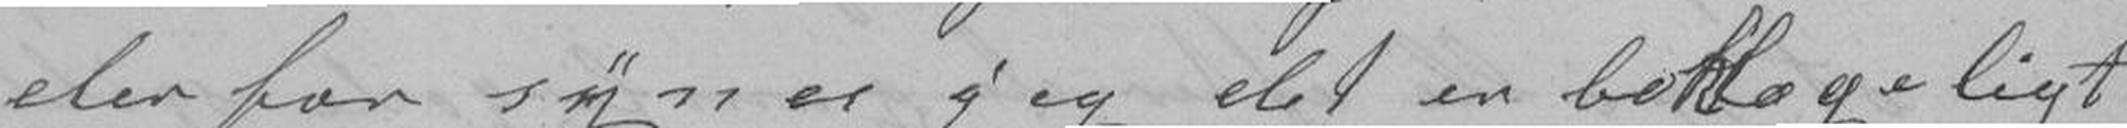derfor synes jeg det er beklageligt
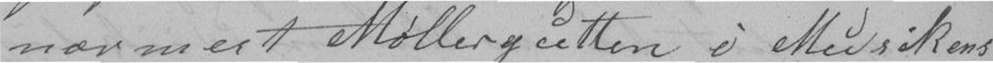nærmest Møllergutten i Musikens
